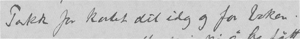Takk for kortet dit idag og for boken.
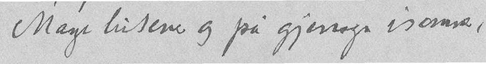Mange hilsener og på gjensyn isommer,
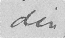din
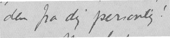den fra dig personlig!
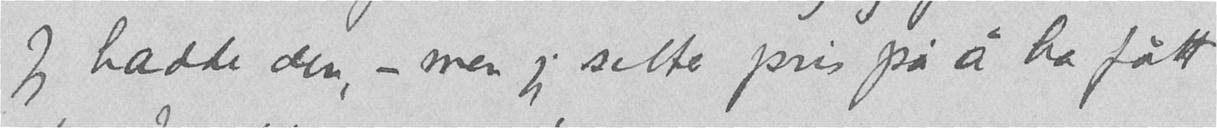Jeg hadde den, – men jeg sitter pris på å ha fått
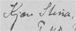Kjære Stina,
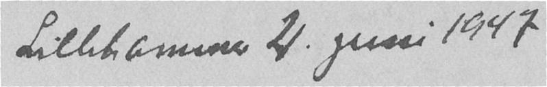Lillehammer 21. juni 1947
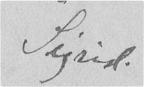Sigrid.
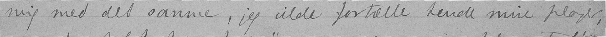mig med det samme, jeg vilde fortælle hende mine plager,
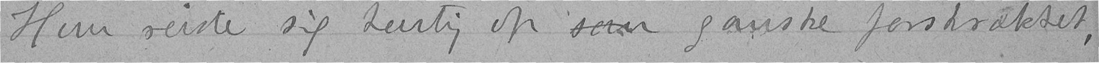Hun reiste sig hurtig op som ganske forskrækket,
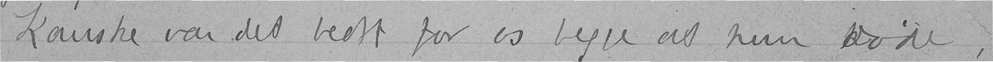Kanske var det bedst for os begge at hun døde,
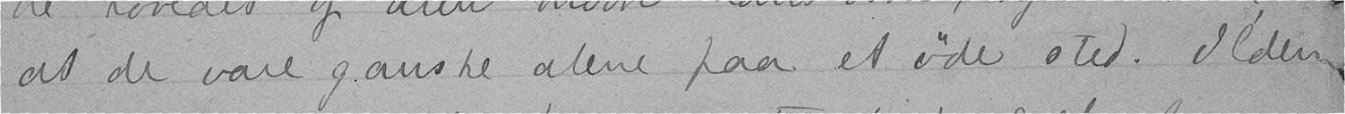at de vare ganske alene paa et øde sted. Ilden
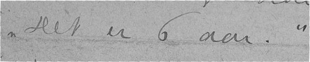"Det er 6 aar."
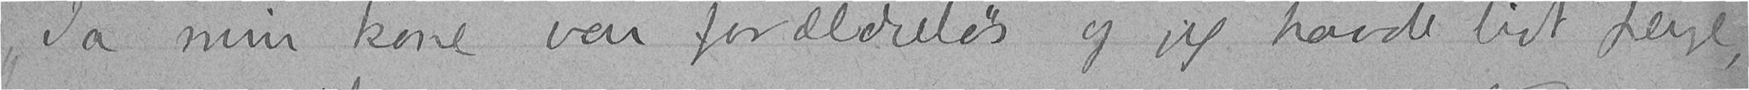"Ja min kone var forældreløs og jeg havde lidt penge,
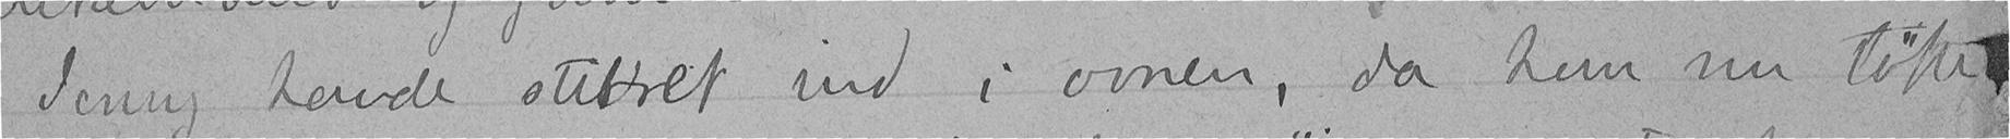Jenny havde stirret ind i ovnen, da hun nu løfte-
